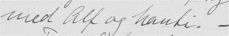med Alf og Nanti. -
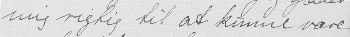mig rigtig til at kunne være
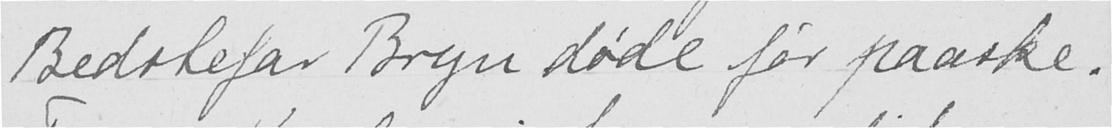Bedstefar Bryn døde før paaske.
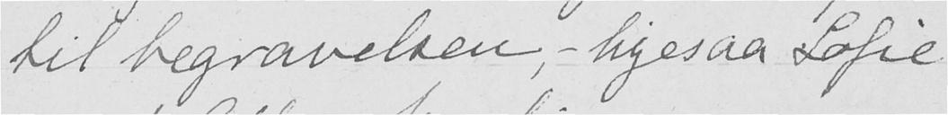til begravelsen - ligesaa Sofie
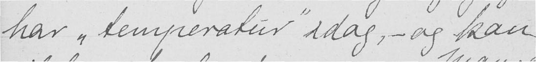har "temperatur" idag,- og kan
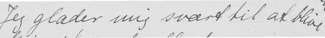Jeg glæder mig svært til at blive
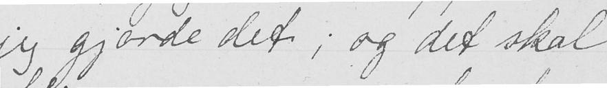jeg gjorde det; og det skal
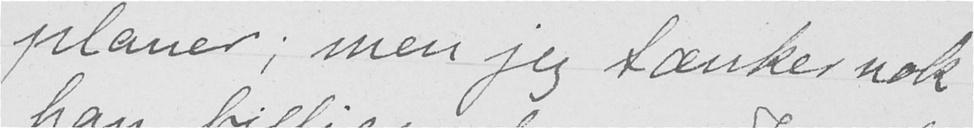planer; men jeg tænker nok
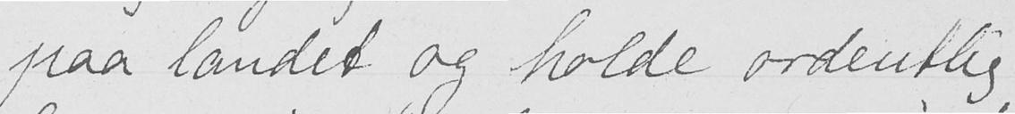paa landet og holde ordentlig
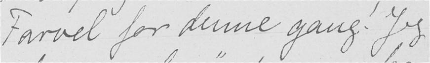Farvel for denne gang! Jeg
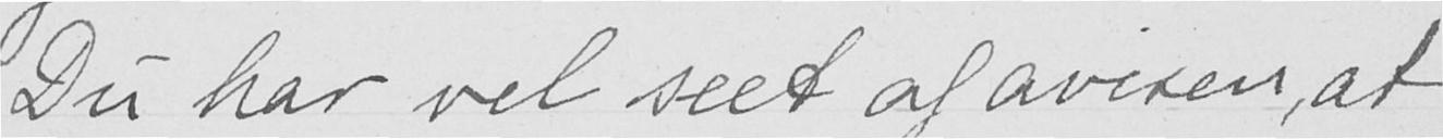Du har vel seet af avisen, at
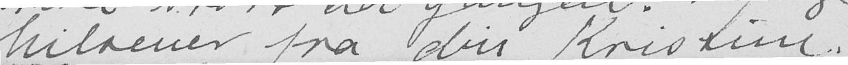hilsener fra din Kristine.
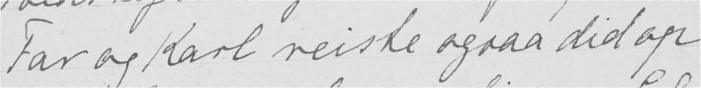Far og Karl reiste ogsaa did op
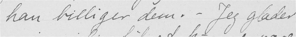han billiger dem. - Jeg glæder
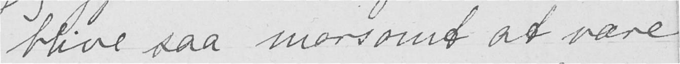blive saa morsomt at være
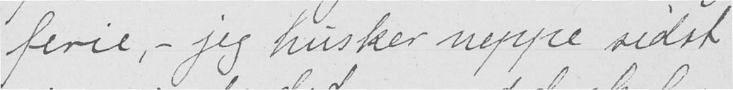ferie, - jeg husker neppe sidst
